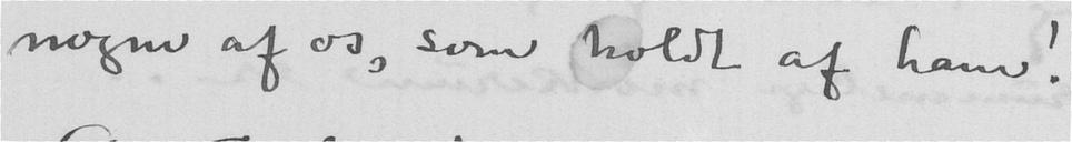nogen af os, som holdt af ham!
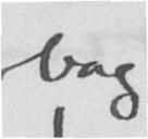bag
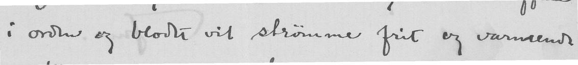i orden og blodet vil strømme frit og varmende
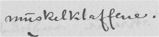muskelklaffene.
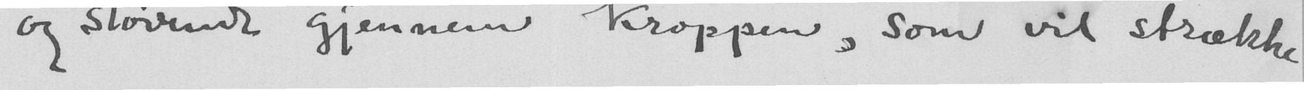og sløvende gjennem kroppen, som vil strække
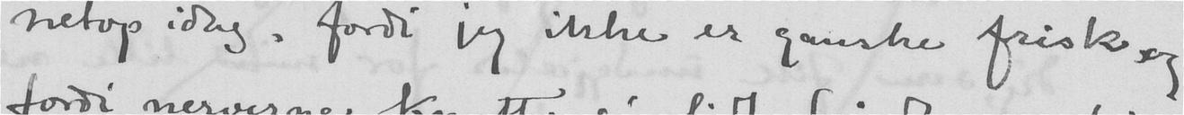netop idag, fordi jeg ikke er ganske frisk, og
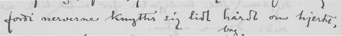fordi nerverne knytter sig lidt hårdt om hjertet
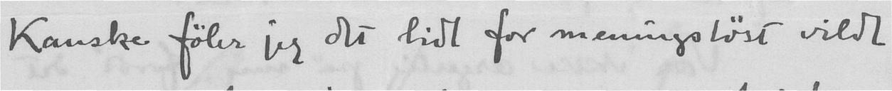Kanske føler jeg det lidt for meningsløst vildt
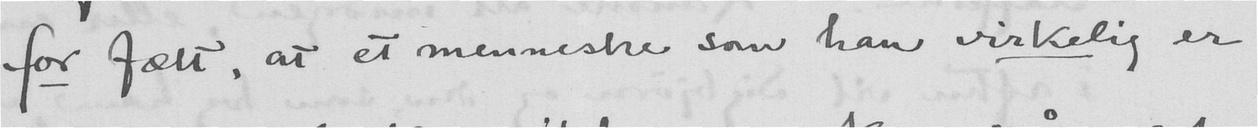for fælt at et menneske som han virkelig er
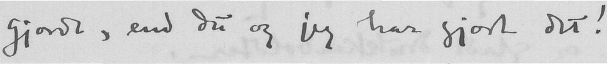gjorde, end du og jeg har gjort det!
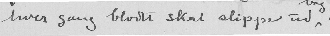hver gang blodet skal slippe ud
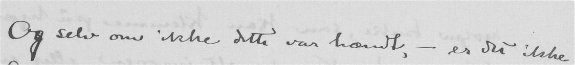Og selv om ikke dette var hændt - er det ikke
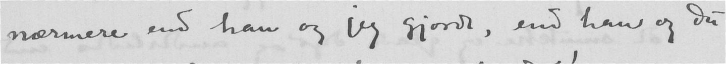nærmere end han og jeg gjorde, end han og du
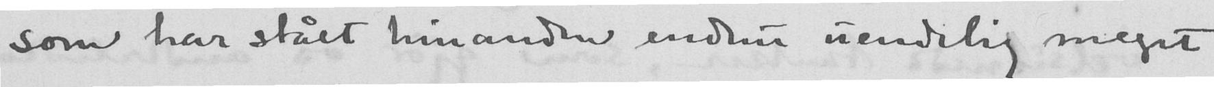som har stået hinanden endnu uendelig meget
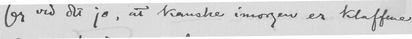Jeg ved det jo, at kanske imorgen er klaffene
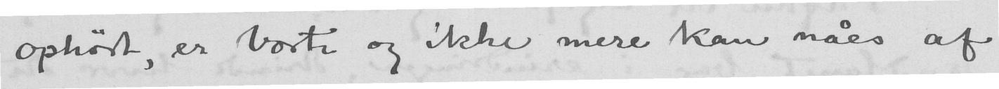ophørt, er borte og ikke mere kan nåes af
